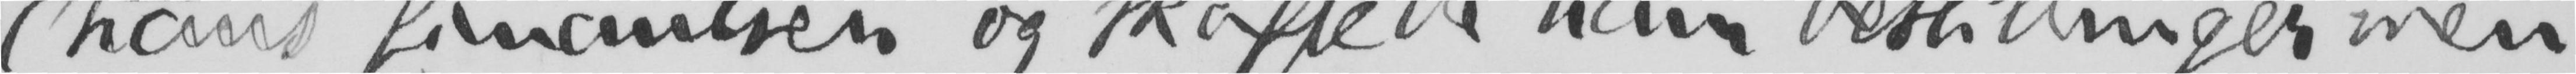hans finantser og skaffede ham bestillinger, men
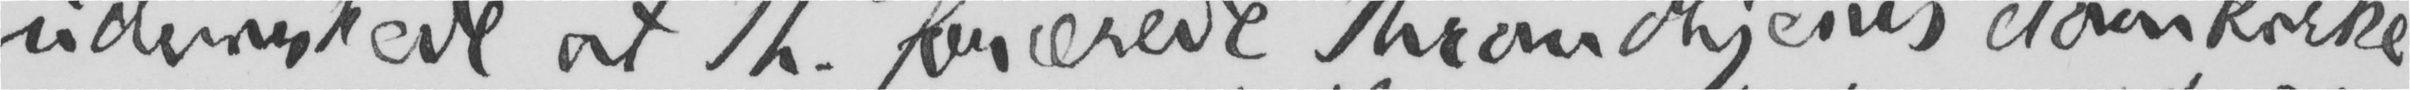udvirkede at Th. forærede Throndhjems domkirke
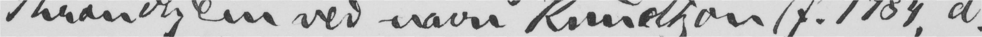Trondhjem ved navn Knudtzon (f. 1784, d.
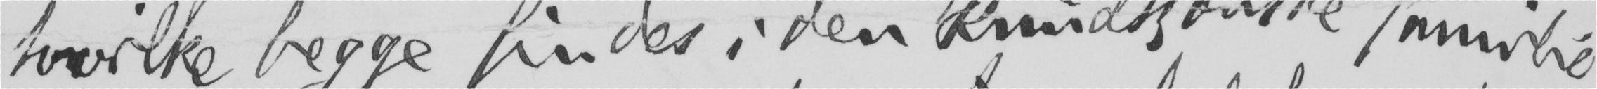hvilke begge findes i den Knudtzonske familie
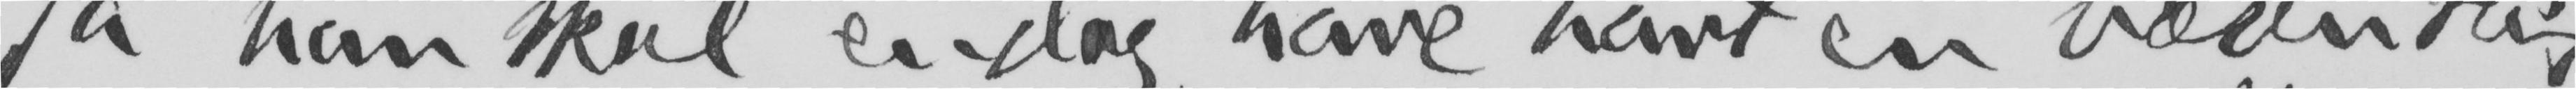ja han skal endog have havt en væsentlig
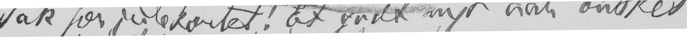Tak for julekortet! Et godt nyt aar ønskes
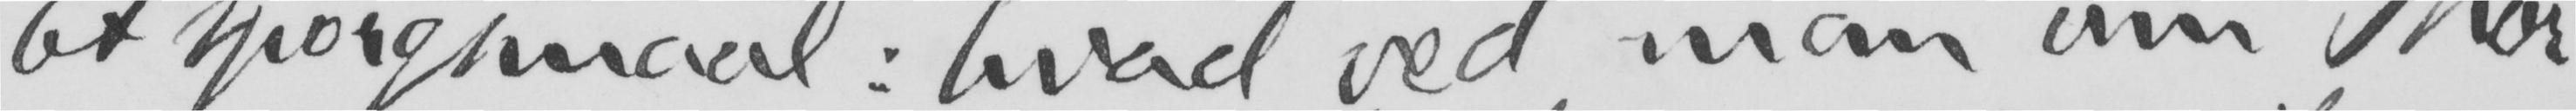Et spørgsmaal: hvad ved man om Thor-
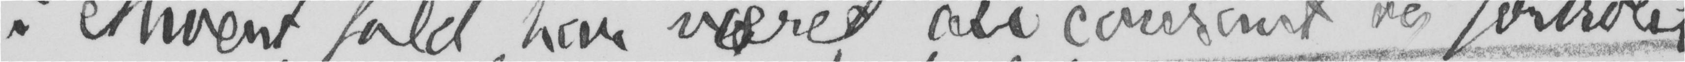i ethvert fald har været au courant og fortrolig
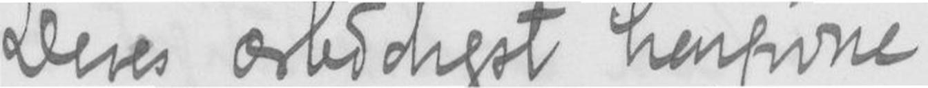Deres ærbødigst hengivne
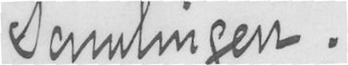samlingen.
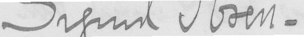Sigurd Ibsen.
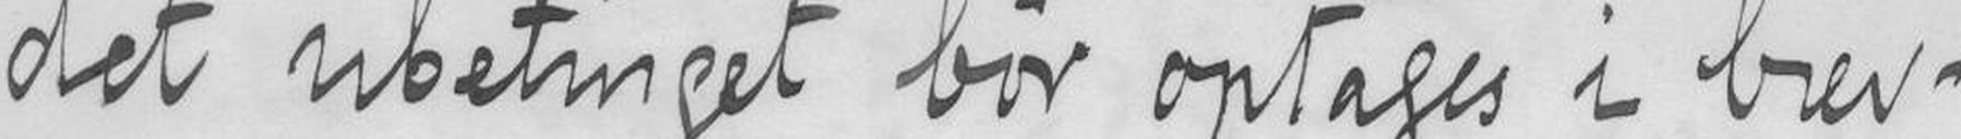det ubetinget bør optages i brev-
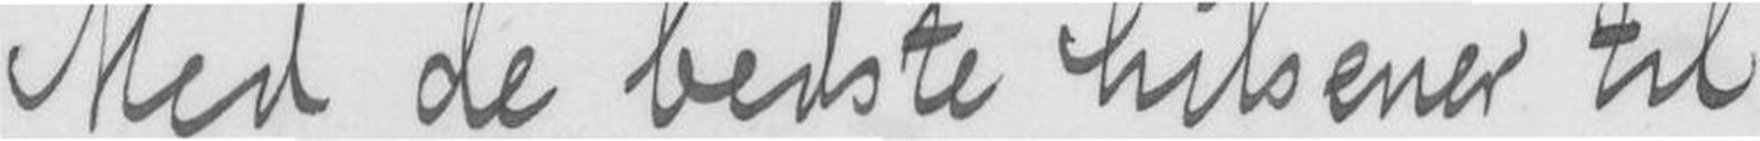Med de bedste hilseener til
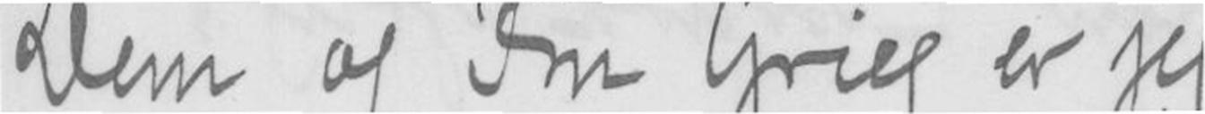Dem og fru Grieg er jeg
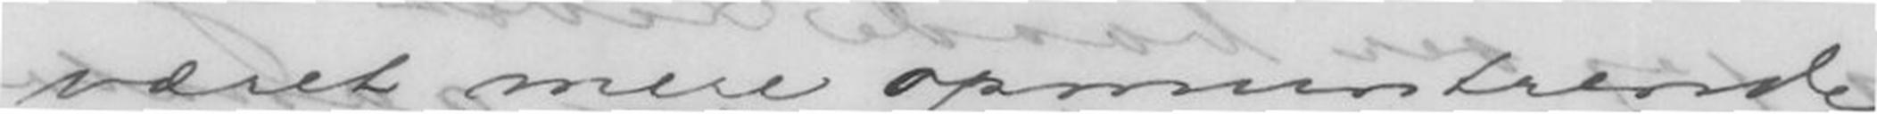været mere opmuntrende,
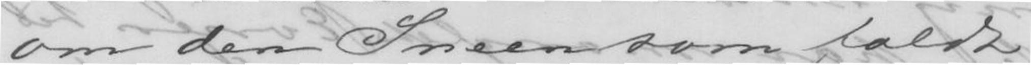om den Sneen som faldt
